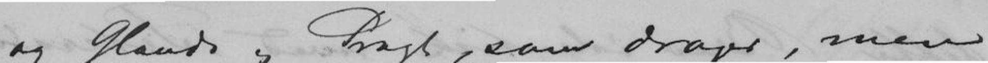og Glands og Pragt, som drager, men
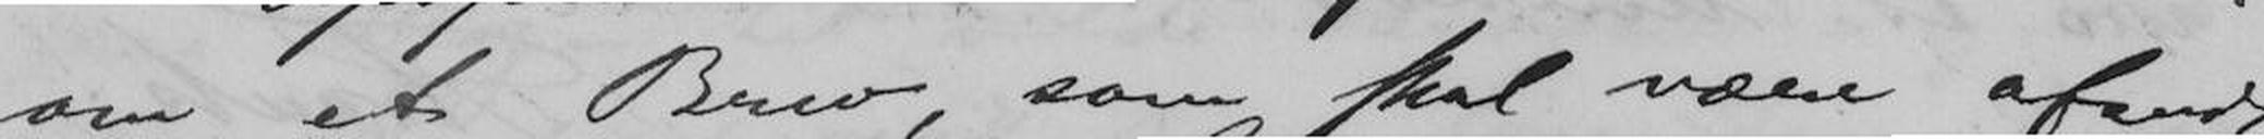om et Brev, som skal være afsendt
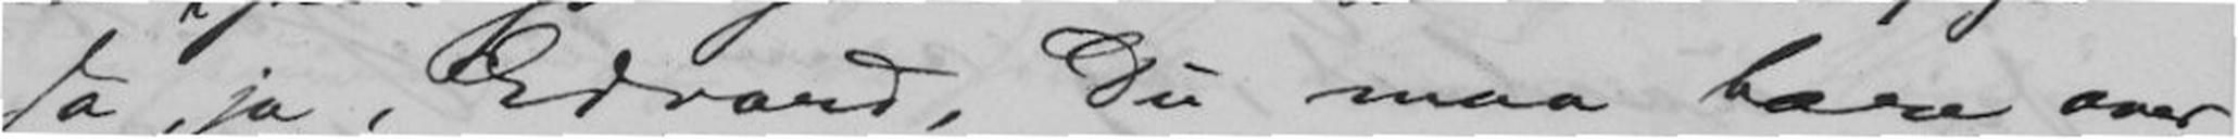Ja, ja, Edvard, Du maa bære over
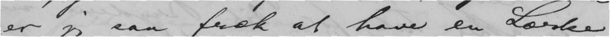er jeg saa fræk at have en Lærke
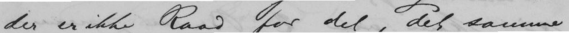der er ikke Raad for det, det samme
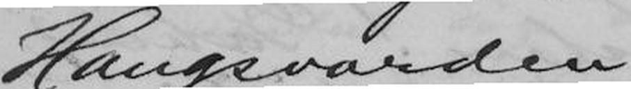Haugsvarden!
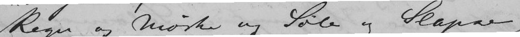Regn og Mørke og Søle og Slapse,
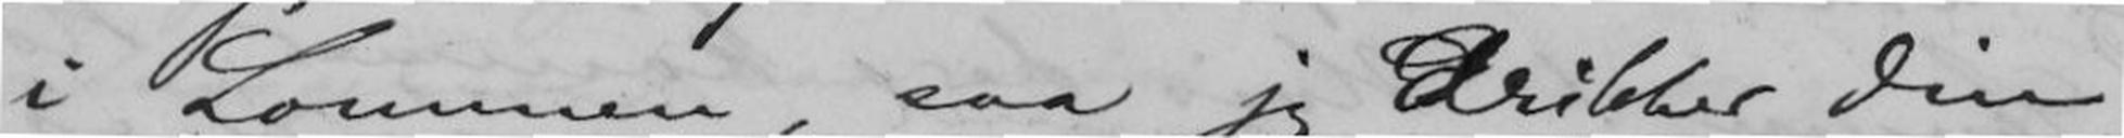i Lommen, saa jeg drikker din
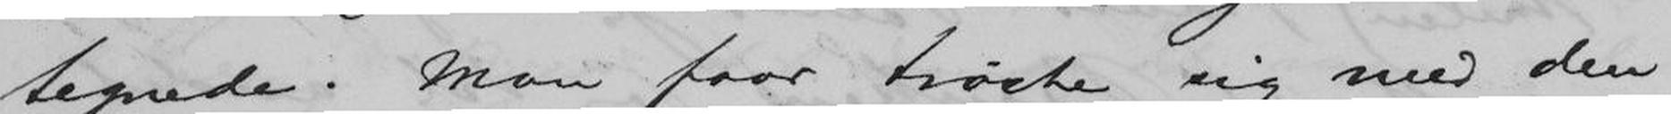tegnede. Man faar trøste sig med den
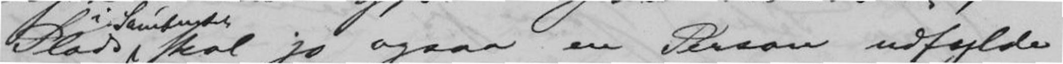Plads i Samfundet, skal jo ogsaa en Person udfylde.
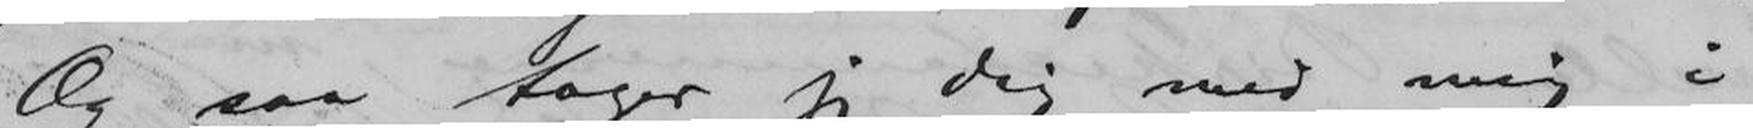Og saa tager jeg dig med mig i
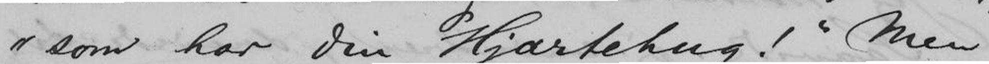"som har din Hjærtehug!" Men
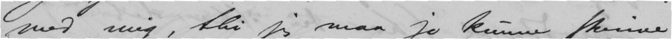med mig, thi jeg maa jo kunne skrive
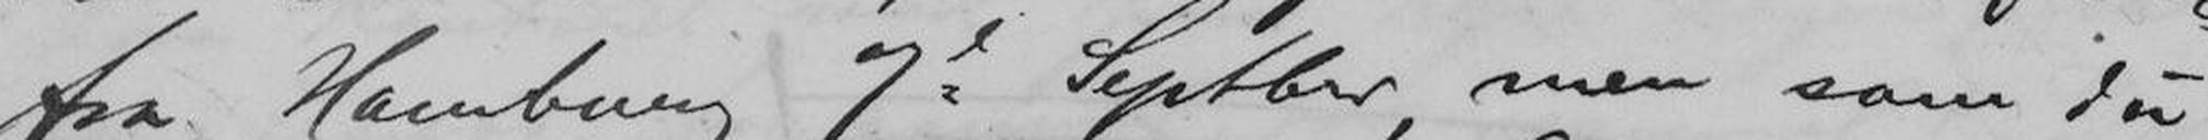fra Hamburg 7de Septbr, men som du
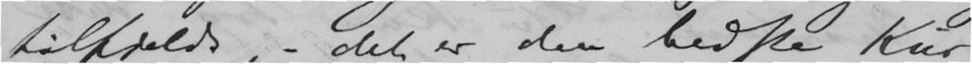tilfjelds, - det er den bedste Kur
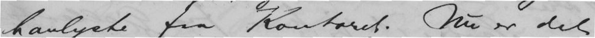banlyste fra Kontoret. Nu er det
